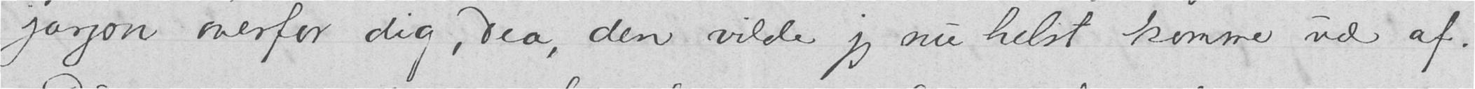jargon overfor dig, Dea, den vilde jeg nu helst komme ud af.
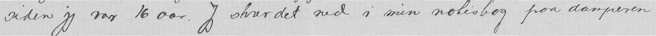siden jeg var 16 aar. Jeg skrev det ned i min notisbog paa damperen
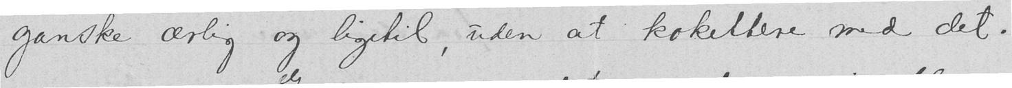ganske ærlig og ligetil, uden at kokettere med det.
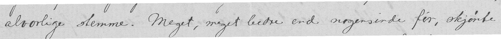alvorlige stemme. Meget, meget bedre end nogensinde før, skjønte
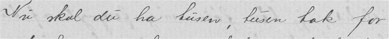Nu skal du ha tusen, tusen tak for
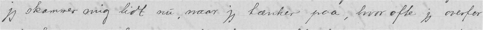jeg skammer mig lidt nu, naar jeg tænker paa, hvor ofte jeg overfor
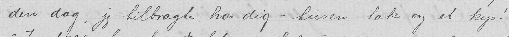den dag, jeg tilbragte hos dig – tusen tak og et kys!
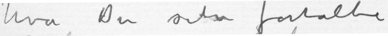hva Du selv fortalte
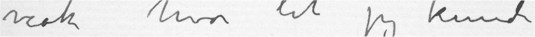nok hvor let jeg kunde
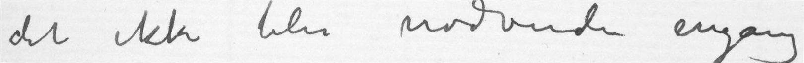det ikke blir nødvendi engang
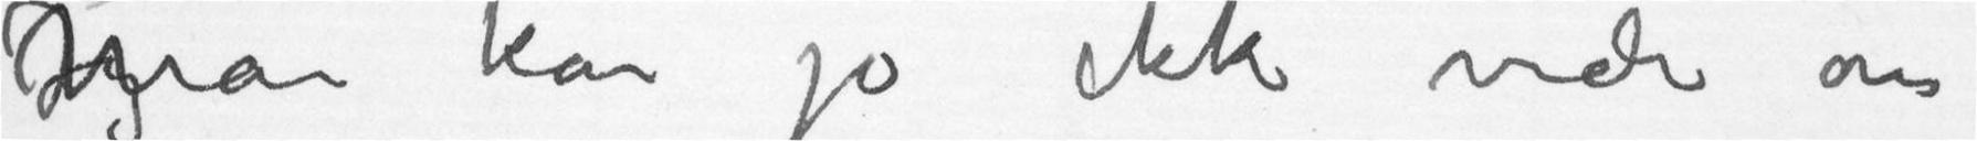JegMan kan jo ikke vide om
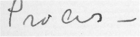Proces –
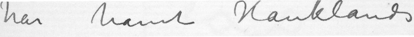har hånet Hauklands
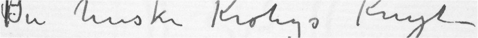Du husker Kroghs Knyt-
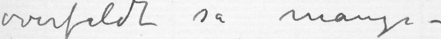overfaldt så mange –
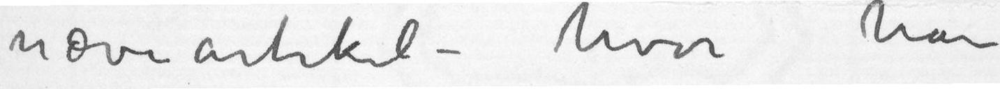næveartikkel – hvor han
